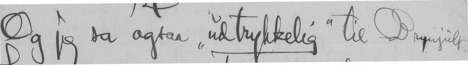Og jeg da ogsaa "udtrykkelig" til Brynjulf
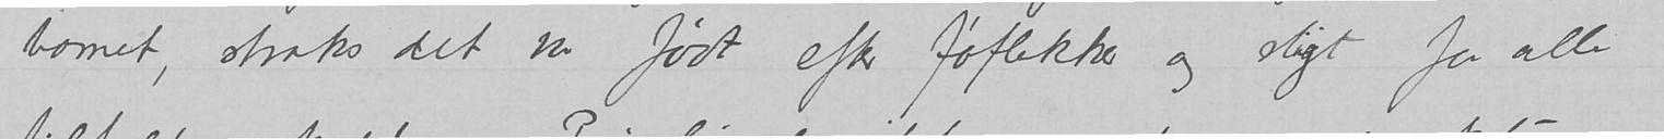barnet, straks det var født efter føflekker og sligt for alle
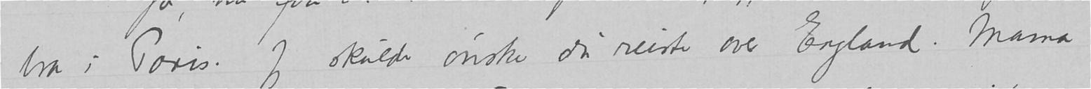bra i Paris. Jeg skulde ønske du reiste over England. Mama
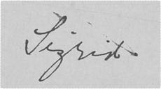Sigrid
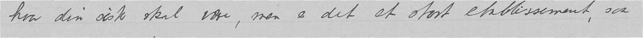hvor din søster skal være, men er det et stort etablissement, saa
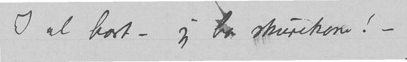I al hast - jeg har skurekone! –
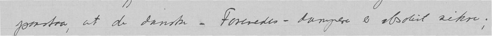paastaar, at de danske-Forenedes-dampere er absolut sikre;
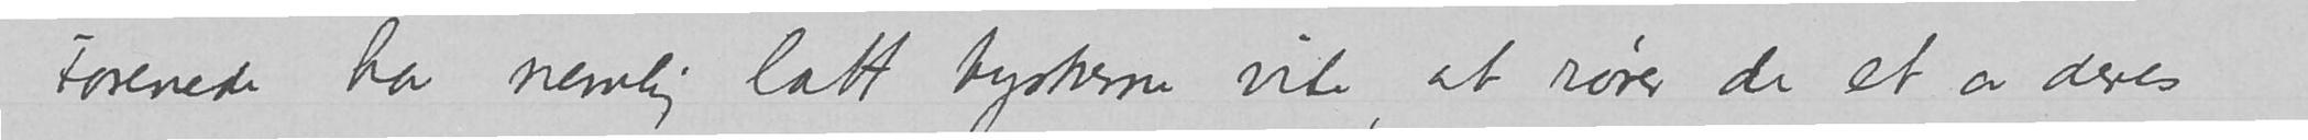Forenede har nemlig latt tyskerne vite at rører de et av deres
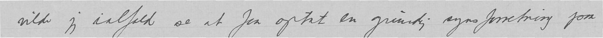vilde jeg ialfald se at faa optat en grundig synsforretning paa
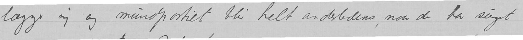lægger sig og mundpartiet blir helt anderledens, naar de har suget
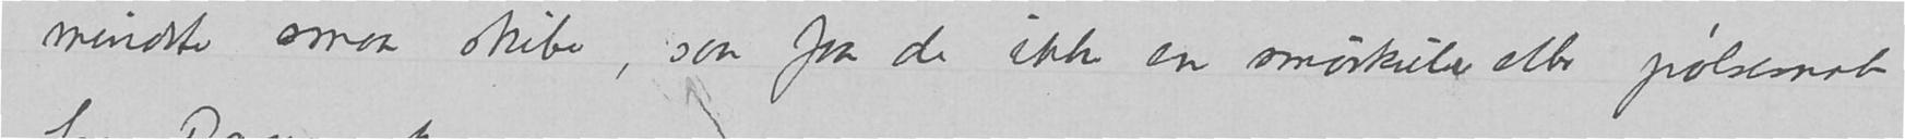mindste smaa skibe, saa faar de ikke en smørkuler eller pølsesnab
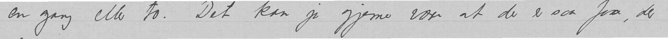en gang eller to. Det kan jo gjerne være at der er saa faa, der
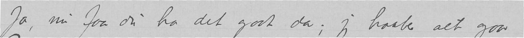Ja, nu faar du har det godt da; jeg haaber alt gaar
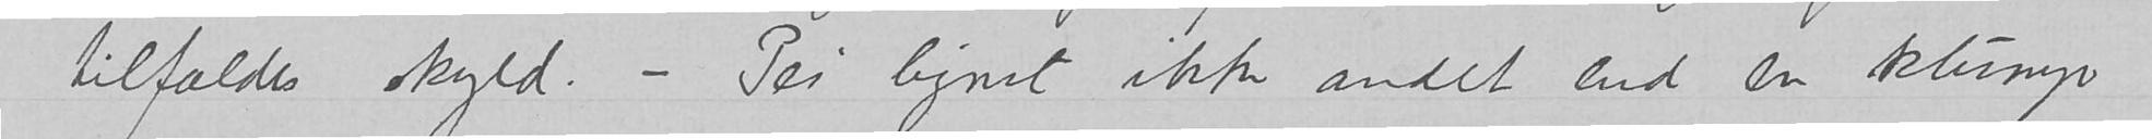tilfældes skyld. – Pei lignet ikke andet end en klump
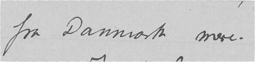fra Danmark mere.
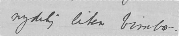nydelig liten bimbo-.
